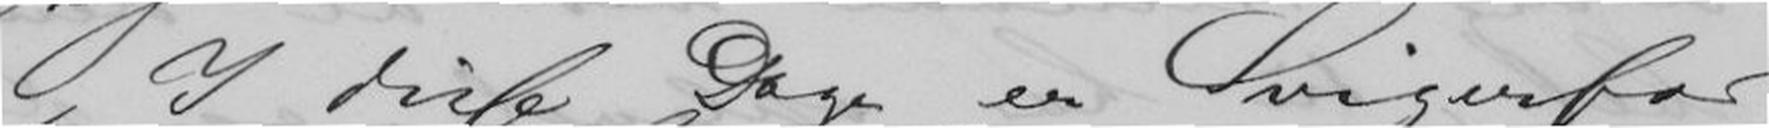I disse Dage er Svigerfar
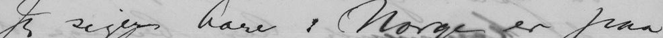Jeg siger bare: Norge er paa
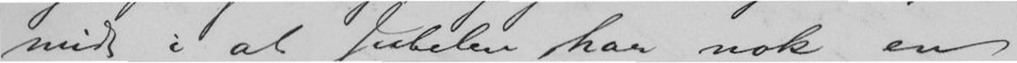at midt i al Jubelen har nok en
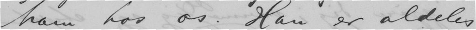ham hos os. Han er aldeles
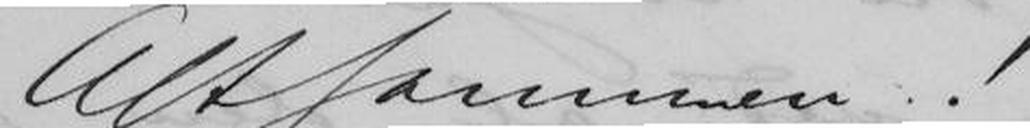Altsammen!
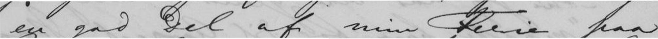en god Del af min Ferie paa
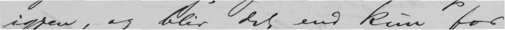igjen, og blir det end kun for
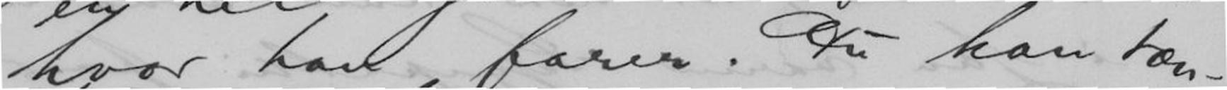hvor han farer. Du kan tæn-
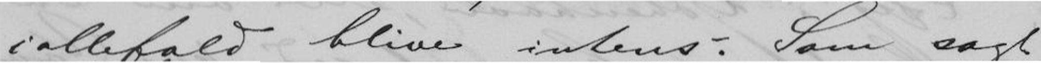ialtfald blive intens. Som sagt
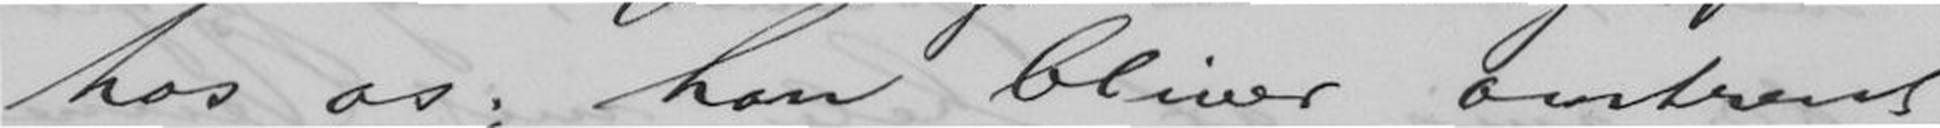hos os; han bliver omtrent
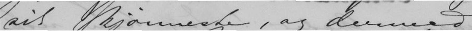sit skjønneste, og dermed
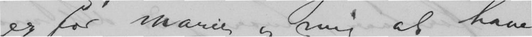er for Marie og mig at have
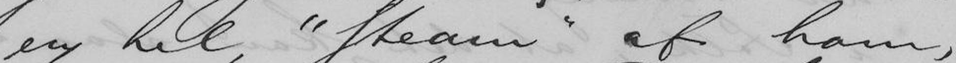en hel "steam" af ham,
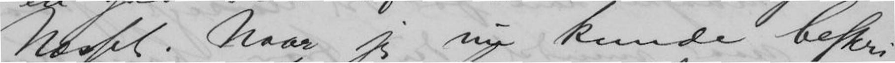Næsset. Naar jeg nu kunde beskri-
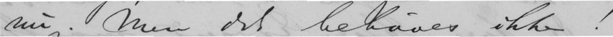nu. Men det behøves ikke!
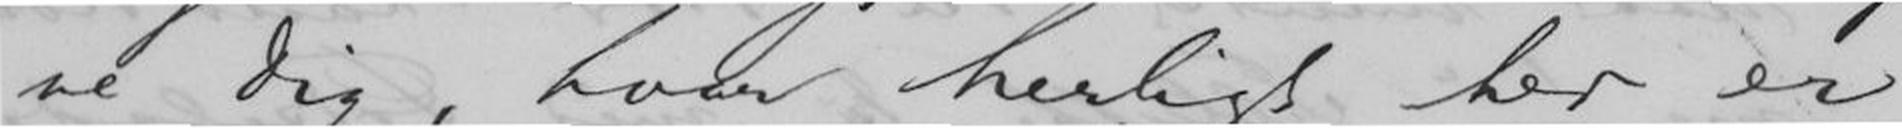ve Dig, hvor herligt her er
